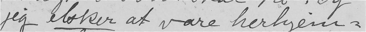jeg elsker at være herhjem-
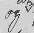og
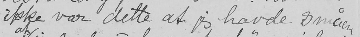ikke var dette at jeg havde småen
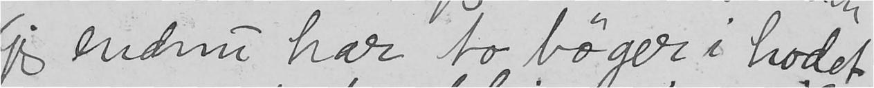og jeg endnu har to bøger i hodet
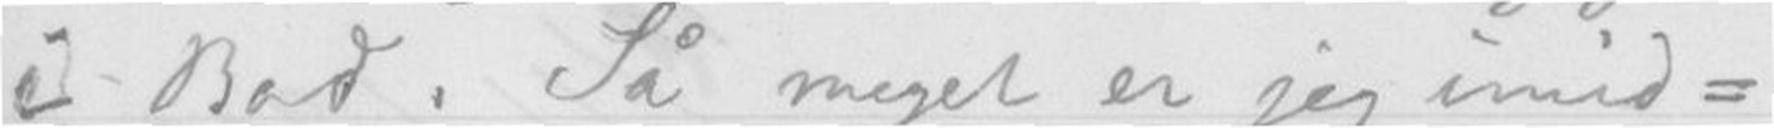i Bad. Så meget er jeg imid-
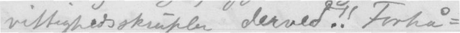vittighedsskrupler derved!! Forhå-
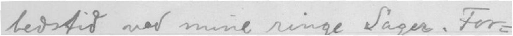bedstid ved mine ringe Sager. For-
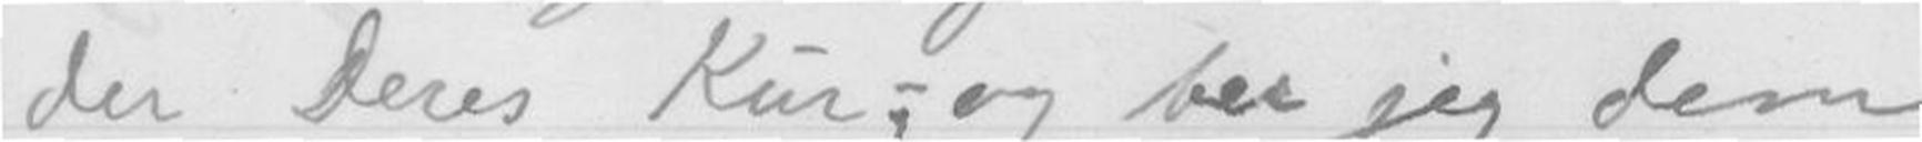der Deres Kur; og ber jeg dem
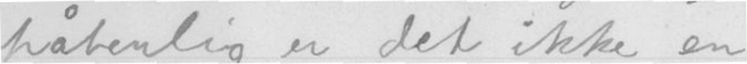håbrenlig er det ikke en
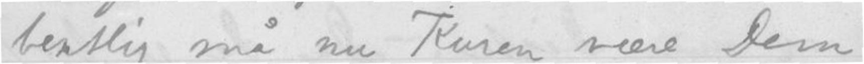bentlig må nu Kuren være Dem
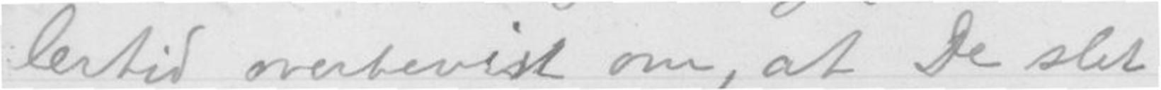lertid overbevist om, at De slet
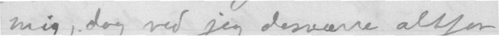mig, dog ved jeg desværre altfor
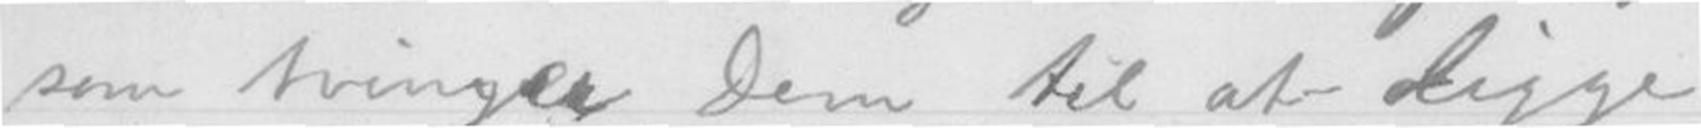som tvinger Dem til at ligge
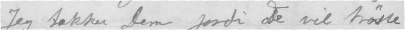Jeg takker Dem fordi De vil trøste
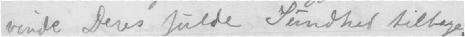vinde Deres fulde Sundhed tilbage.
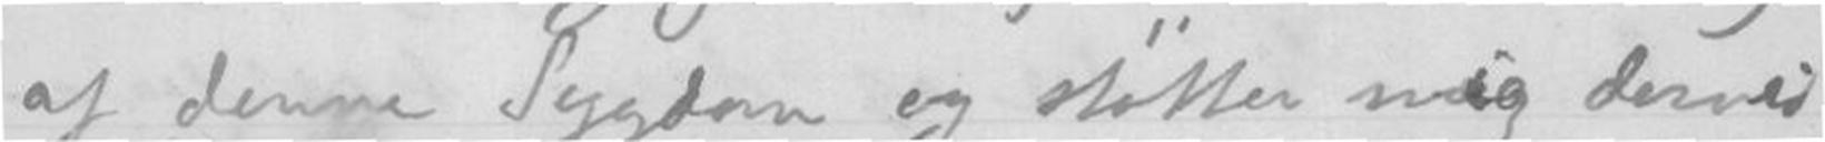af denne Sygdom og støtter mig derved
